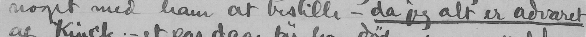noget med ham at bestille - da jeg alt er advaret
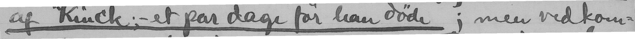af Kinck; - et par dage før han døde ; men vedkom-
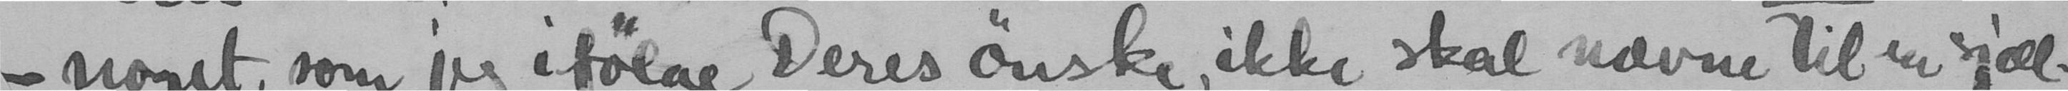- noget, som jeg ifølge Deres ønske, ikke skal nævne til en sjæl-
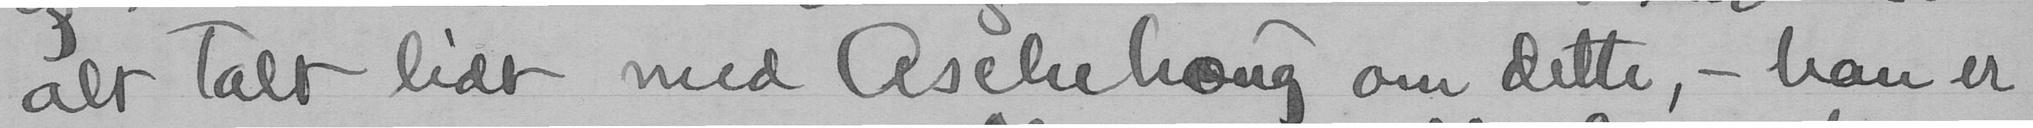alt talt lidt med Aschehaug om dette, - han er
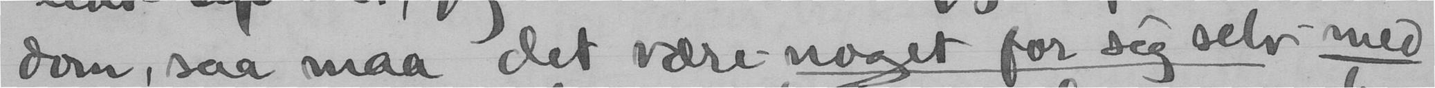dom, saa maa det være noget for sig selv med
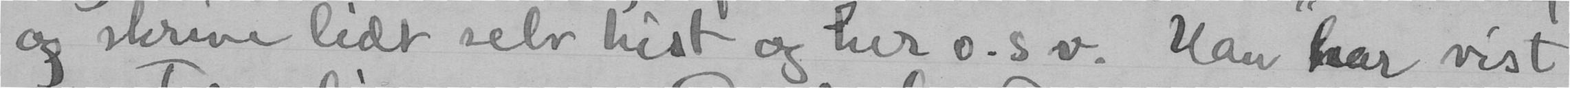og skrive lidt selv hist og her o.s.v. Han har vist
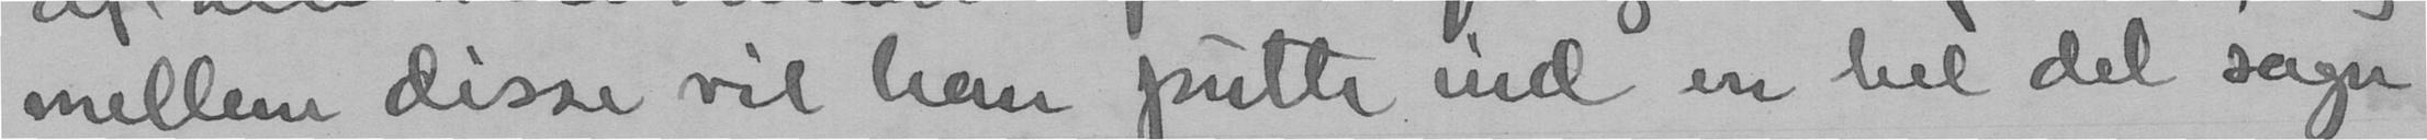mellem disse vil han putte ind en hel del sagn
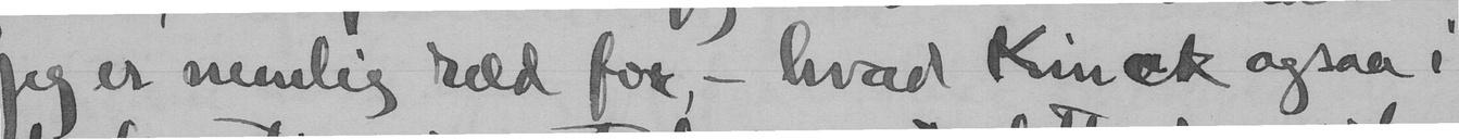Jeg er nemlig ræd for - hvad Kinck ogsaa i
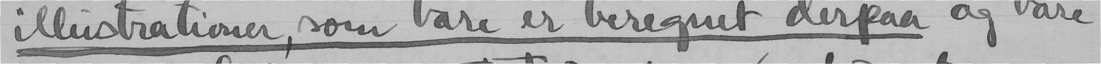illustrationer, som bare er beregnet derpaa og bare
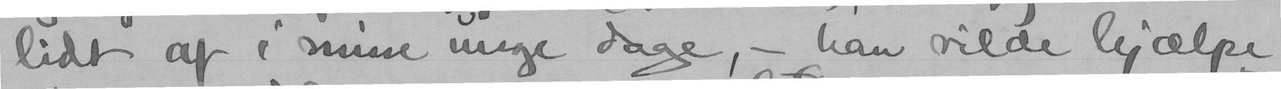lidt af i mine unge dage, - han vilde hjælpe
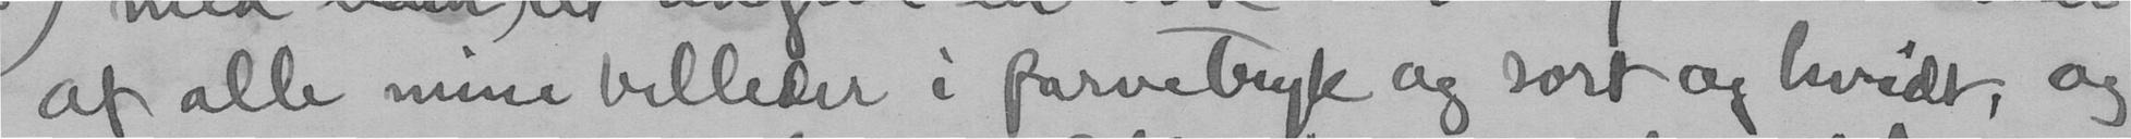af alle mine billeder i farvetryk og sort og hvidt, og
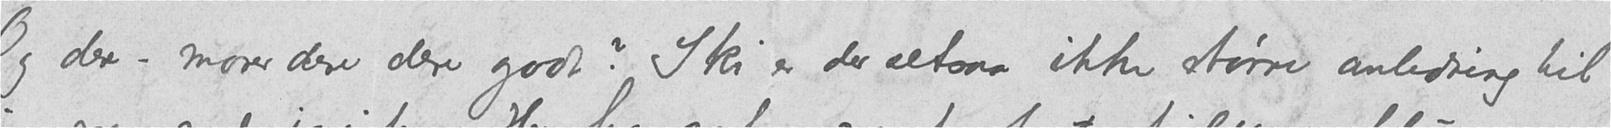Og dere – morer dere dere godt? Ski er der altsaa ikke større anledning til
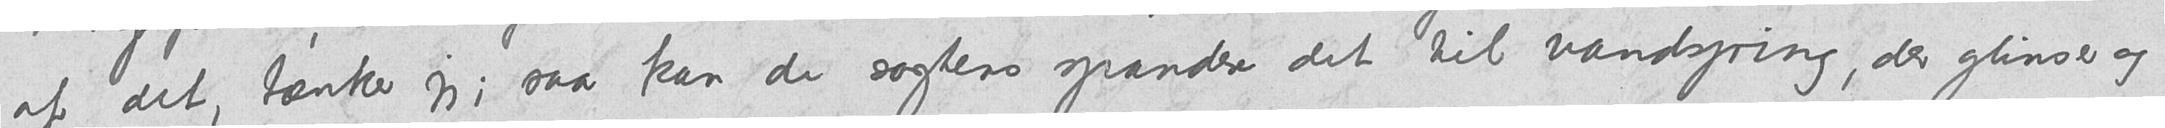af det, tænker jeg; saa kan de sagtens spandere det til vandspring, der glinser og
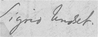Sigrid Undset.
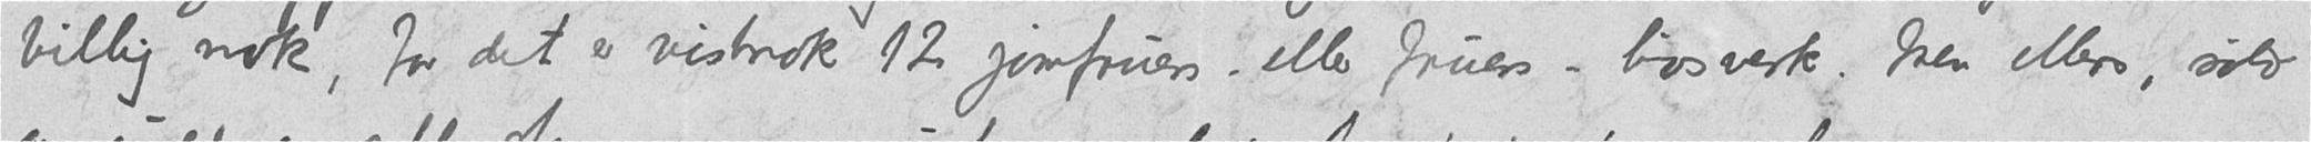billig nok, for det er vistnok 12 jomfruers – eller fruers – livsverk. Men ellers, sølv
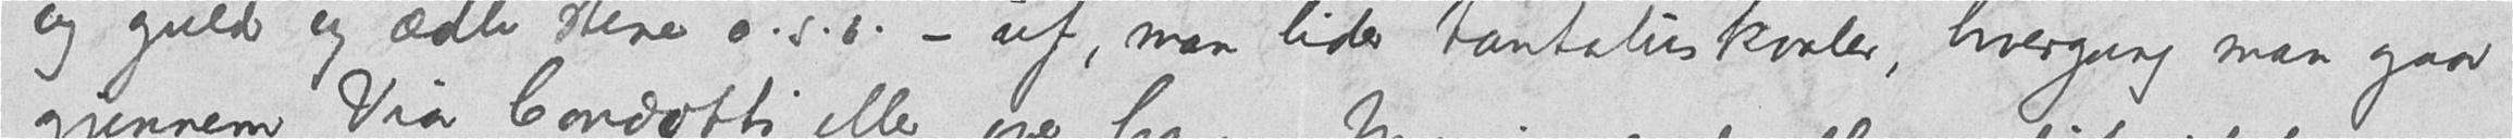og guld og ædle stene o.s.v. - uf, man lider tantaluskvaler, hvergang man gaar
